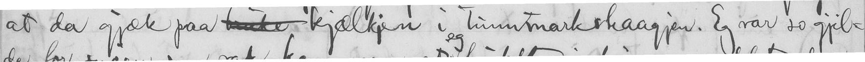at da gjæk paa  kjælkjen i tunnmarkshaagjen. Eg var so gjel-
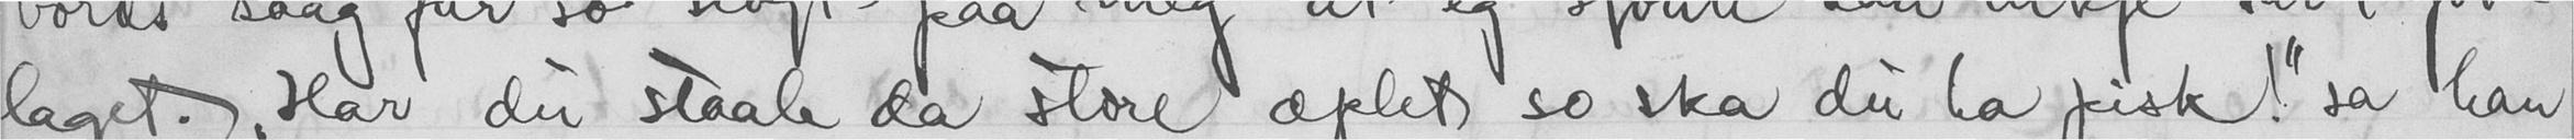laget. "Har du staale da støre æplet so ska du ha pisk!" sa han.
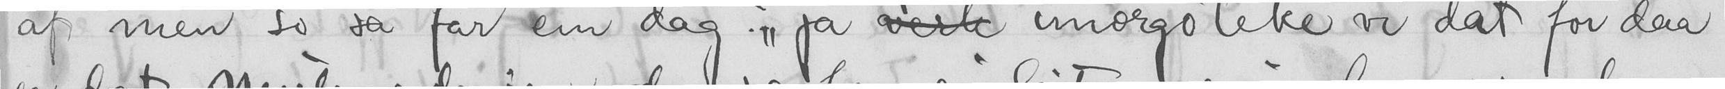af men so sa far ein dag: "ja  imorgo teke vi dat for daa
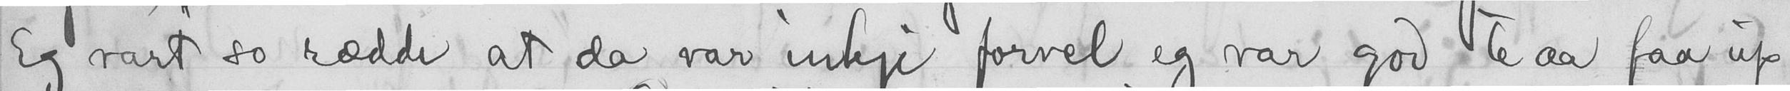Eg vart so rædde at da var inkje forvel og var god te aa faa up
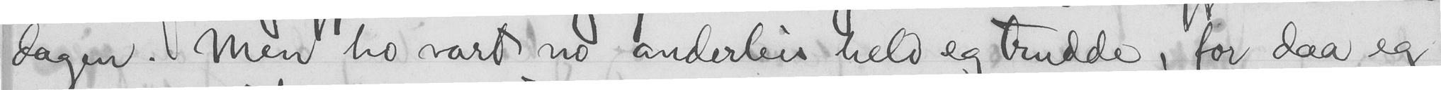dagen. Men ho vart no anderleis held eg trudde, for daa eg
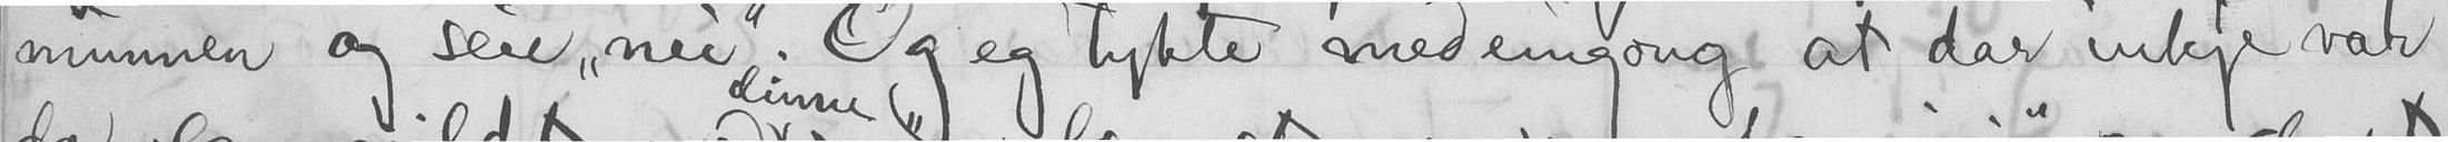munnen og seie "nei". Og eg tykte med eingong at dar inkje var
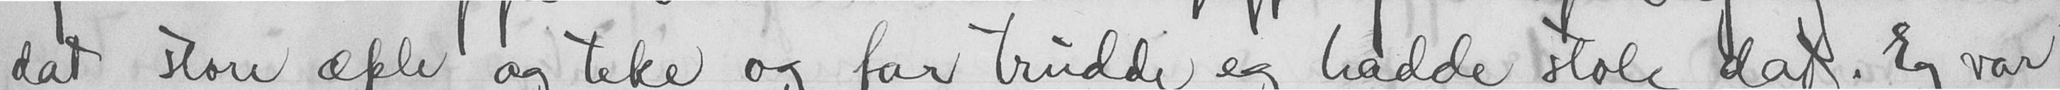dat store æple og teke og far trudde eg hadde stole dat. Eg var
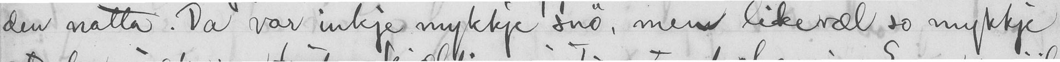den natta. Da var inkje mykkje snø, men likevæl so mykkje
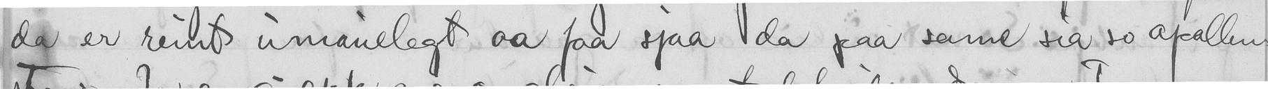da er reint umauelegt aa faa sjaa da paa same sia so apallen
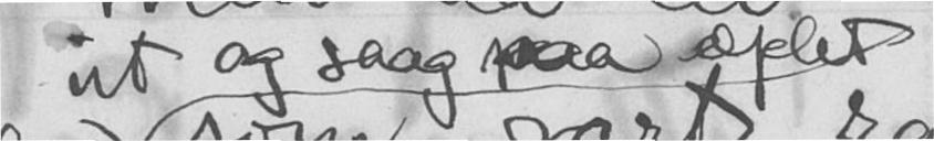ut og saag paa æplet
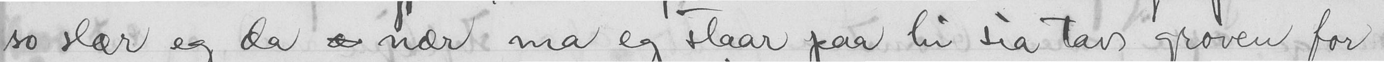so slær og da  nært ma eg slaar paa sia tav groven for
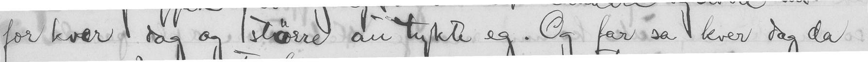for kver dag og større au tykte eg. Og far sa kver dag da
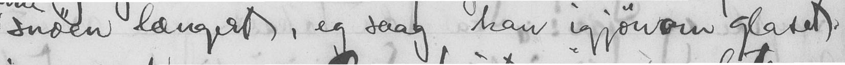snøen længest, eg saag han igjønom glaset.
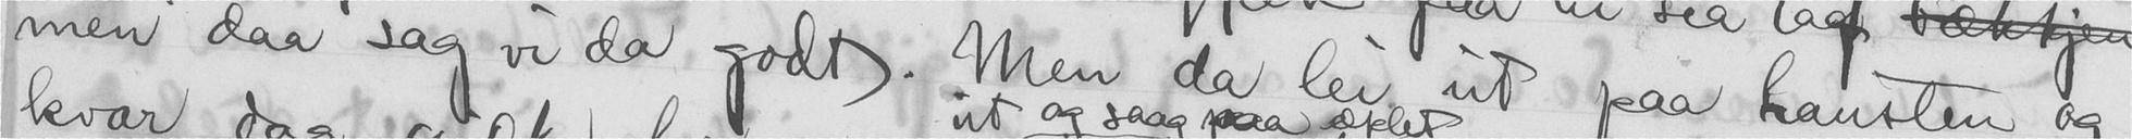men daa sag vi da godt. Men da lei ut paa hansten og
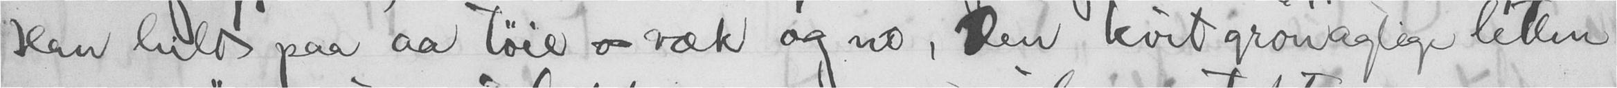Han hilt paa aa tøie o væk og no, den kvitgrønagtige letlen
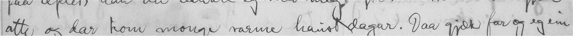atte og dar kom monge varme haustdagar. Daa gjæk far og eg ein
